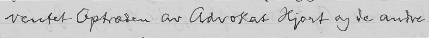ventet Optræden av Advokat Hjort og de andre
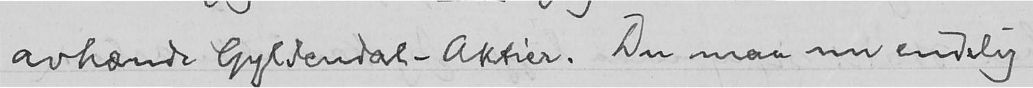avhænde Gyldendal-Aktier. Du maa nu endelig
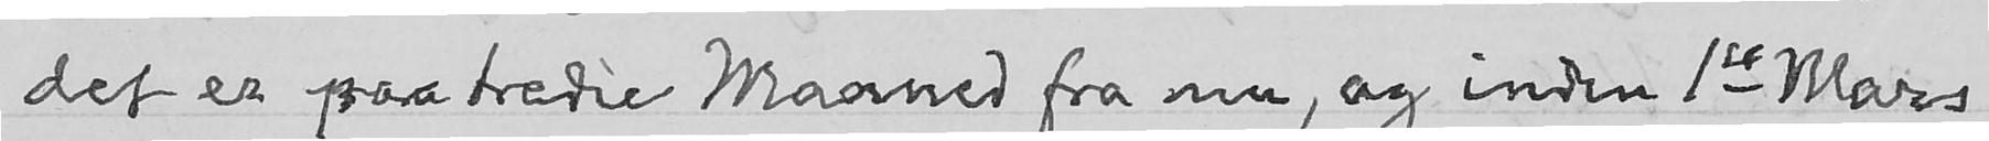det er paa tredie Maaned fra nu, og inden 1st Mars
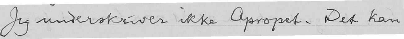Jeg underskriver ikke Opropet. Det kan
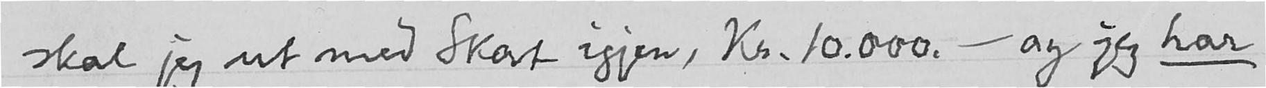skal jeg ut med Skat igjen, Kr. 10.000. - og jeg har
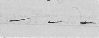- - -
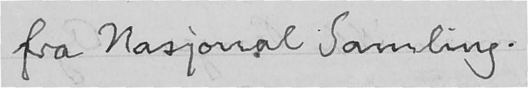fra Nasjonal Samling.
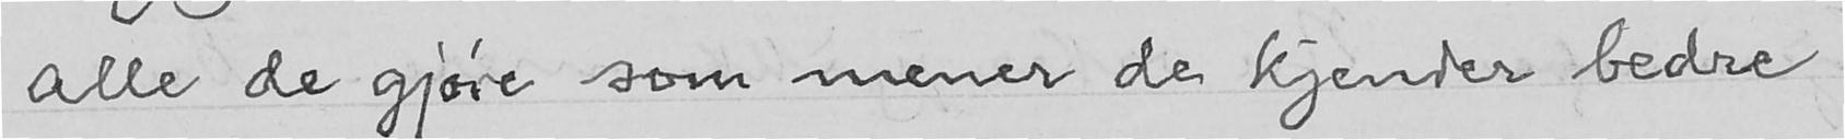alle de gjøre som mener de kjender bedre
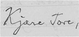Kjære Tore,
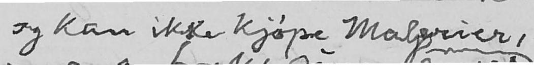og kan ikke kjøpe Malerier,
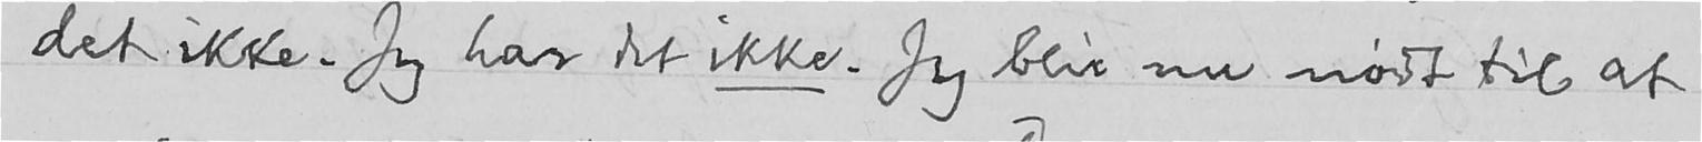det ikke. Jeg har det ikke. Jeg blir nu nødt til at
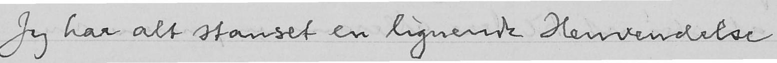Jeg har alt stanset en lignende Henvendelse
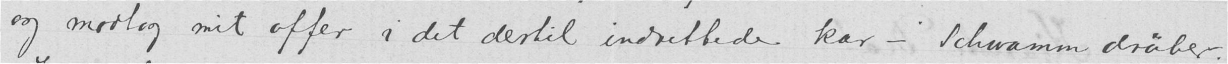og modtog mit offer i det dertil indrettede kar - schwamm drüber.
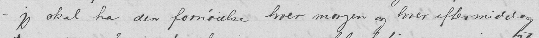- jeg skal ha den fornøielse hver morgen og hver eftermiddag
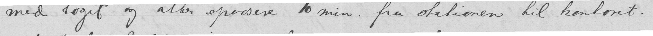med toget og atter spadsere 10 min. fra stationen til kontoret.
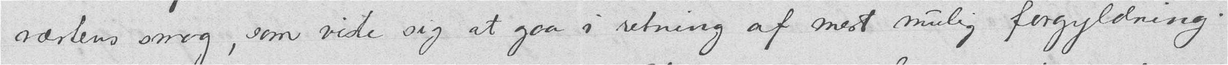værtens smag, som viste sig at gaa i retning af mest mulig forgyldning.
